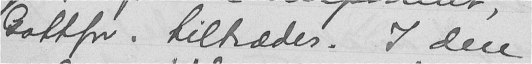Gottfr. tiltræder. I den
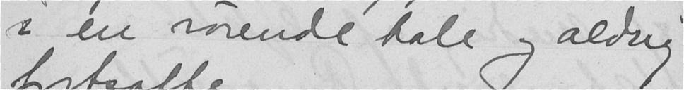i en rørende tale og aldrig
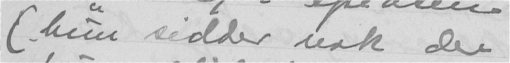(hun sidder nok der
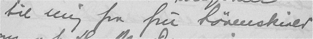til mig fra fru Løvenskiold
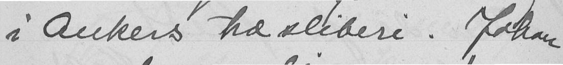i Ankers træsliberi. Johan
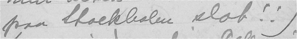paa Stockholm slot!!)
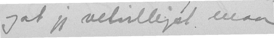og at jeg velvilligst maa
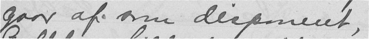gaar af som disponent,
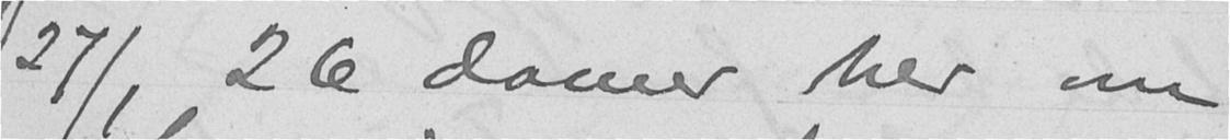27/1 26 damer her om
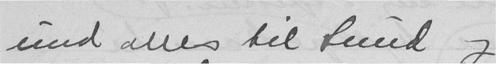und alles til Sund og
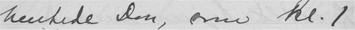hentede Don, som kl. 1
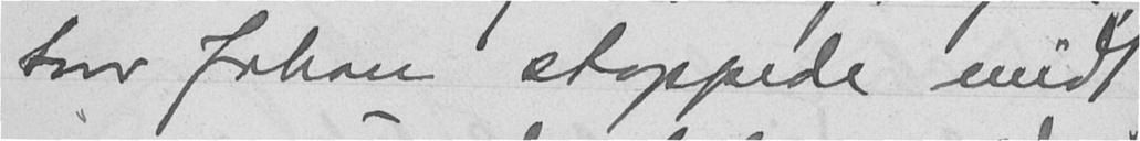hvor Johan stoppede midt
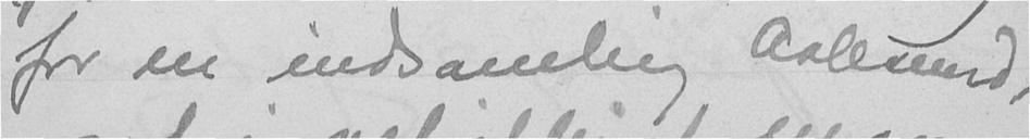for en indsamling Aalesund,
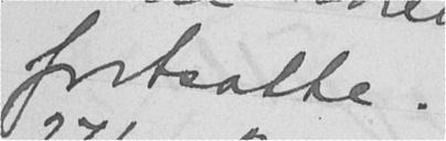fortsatte.
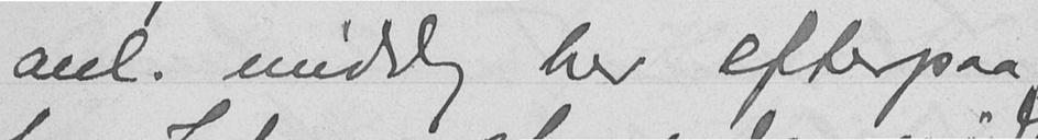anl. middag her efterpaa
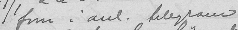form i anl. telegram
